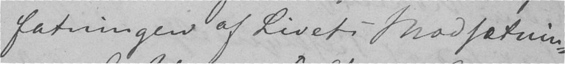fatningen af Livets Modsætnin-
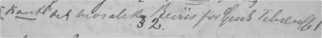(Kant det moralske Beviis for Guds Tilværelse)
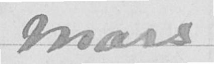Mars
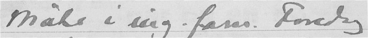Mate i iig. forn. Forsdag
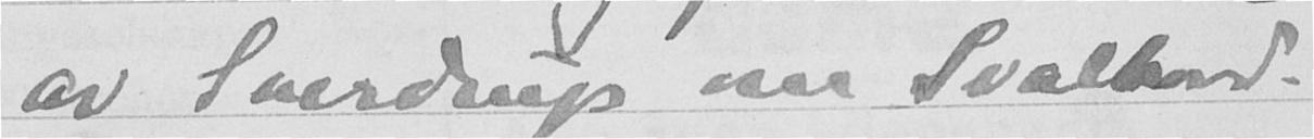at Sverdrup om Svalband.
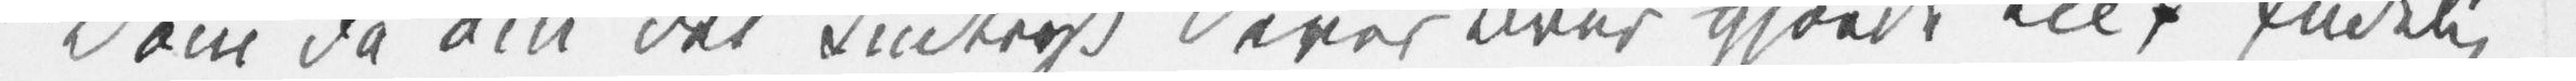Døm da om det Indtryk Deres Brev gjorde Lie. Endelig
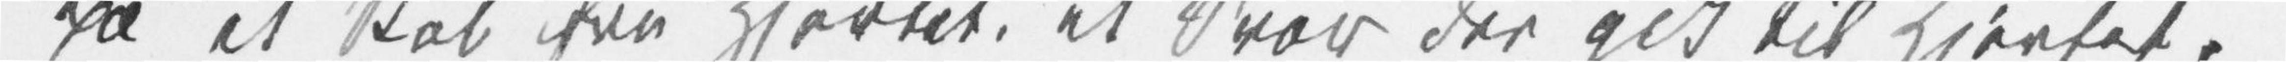på et Råb fra Hjertet, et Svar der gik til Hjertet.
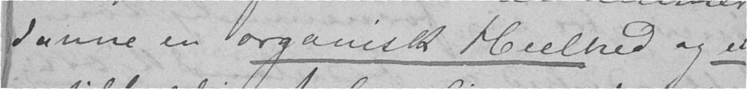danne en organisk Heelhed og ei
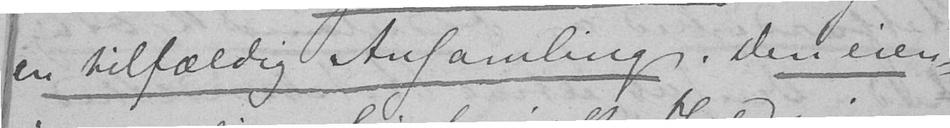en tilfældig Ansamling. Den eien-
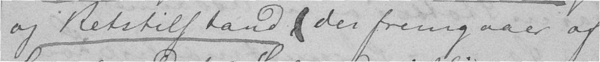og Retstilstand (der fremgaaer af
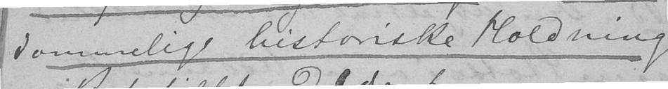dommelige historiske Holdning
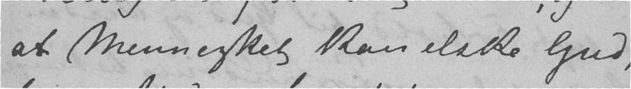at Mennesket kan elske Gud,
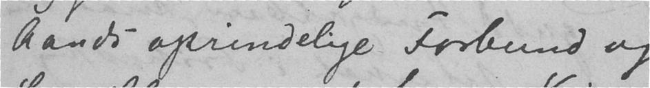Aands oprindelige Forbund og
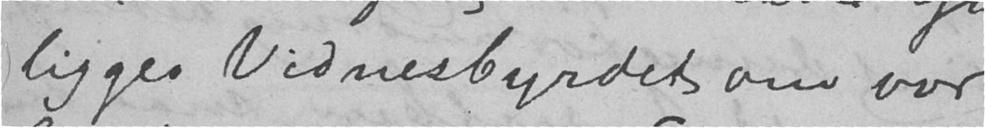ligger Vidnesbyrdet om vor
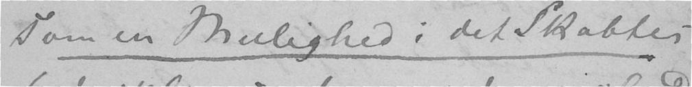som en Mulighed i det Skabtes
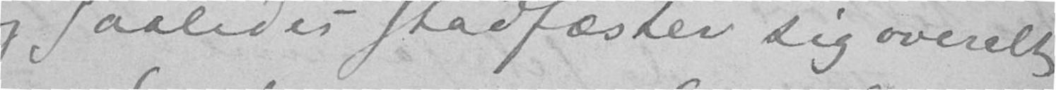og saaledes stadfæster sig overalt
